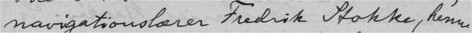navigationslærer Fredrik Stokke, henne
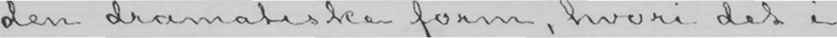den dramatiske form, hvori det i
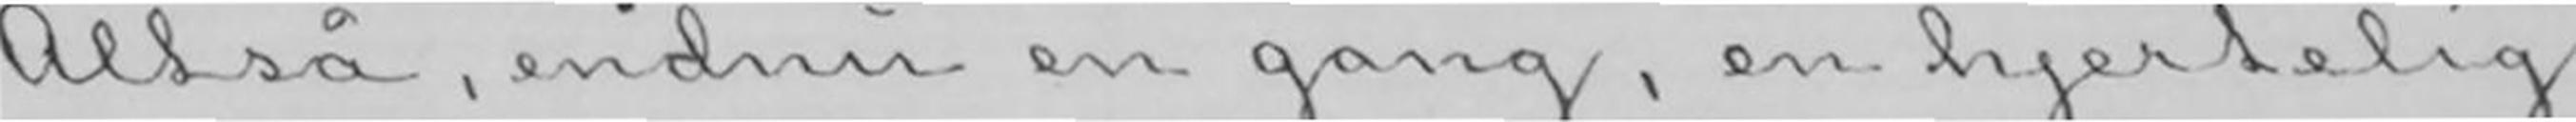Altså, endnu en gang, en hjertelig
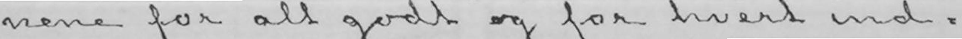nene for alt godt og for hvert ind-
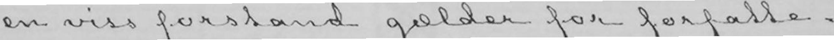en viss forstand gælder for forfatte-
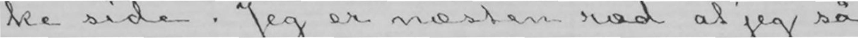ke side. Jeg er næsten ræd at jeg så
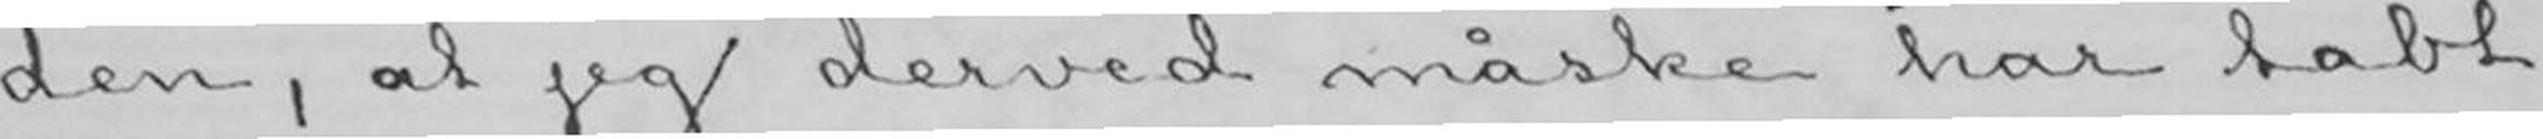den, at jeg derved måske har tabt
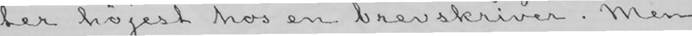ter højest hos en brevskriver. Men
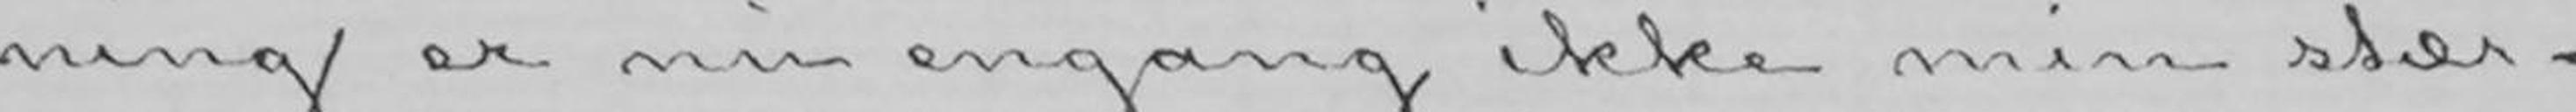ning er nu engang ikke min stær-
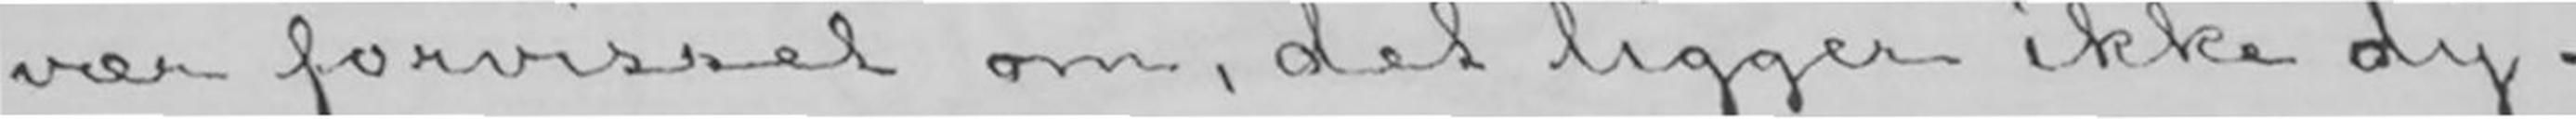vær forvisset om, det ligger ikke dy-
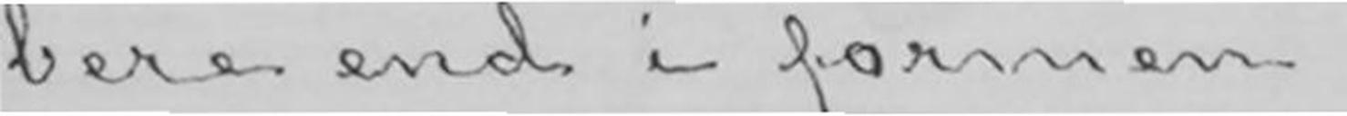bere end i formen.
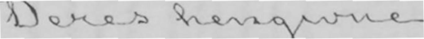Deres hengivne
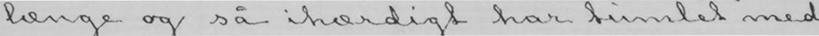længe og så ihærdigt har tumlet med
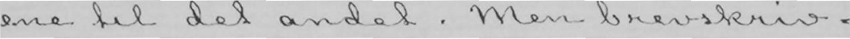ene til det andet. Men brevskriv-
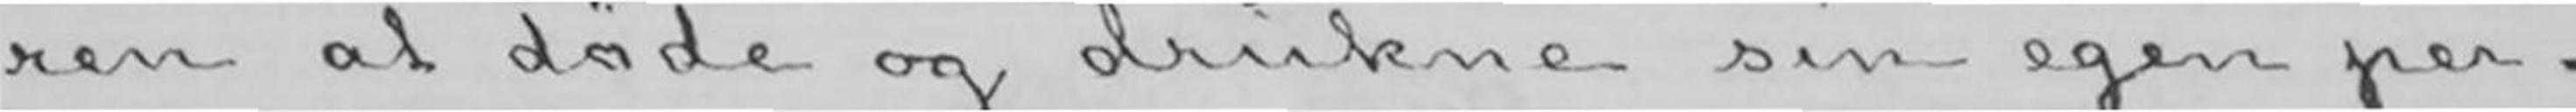ren at døde og drukne sin egen per-
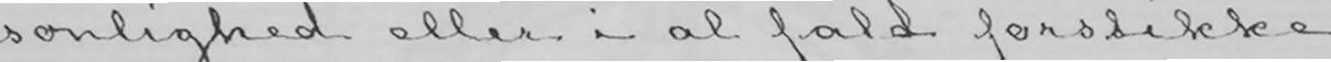sonlighed eller i al fald forstikke
